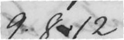9.8.12
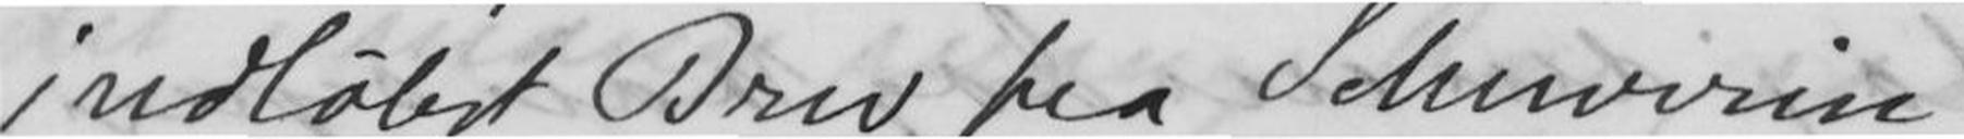indløbet Brev fra Schwerin
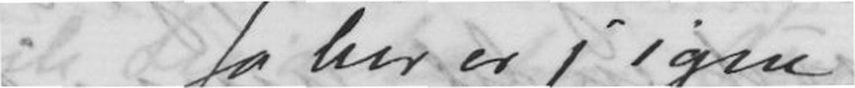Ja her er j igen
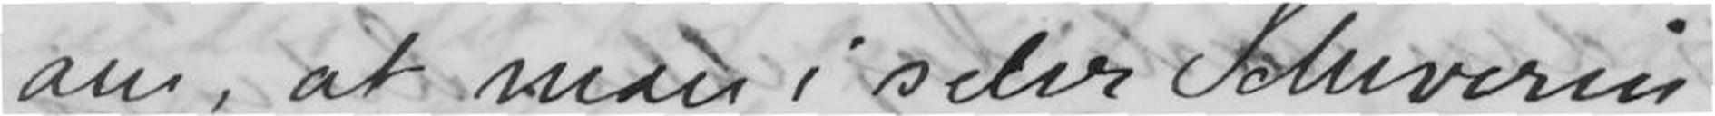om, at man i selve Schwerin
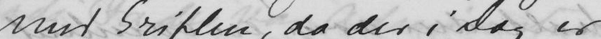med Griflen, da der i Dag er
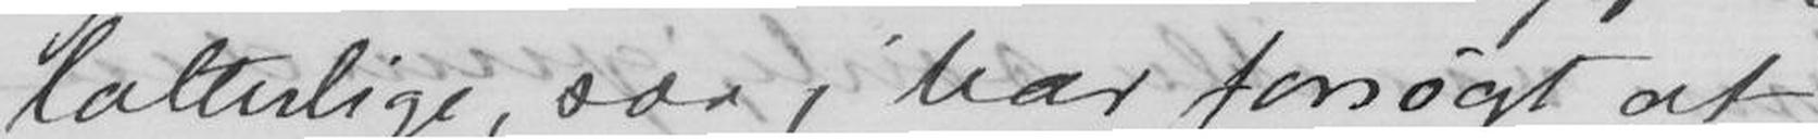latterlige, saa j har forsøgt at
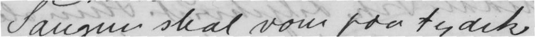Sangen skal vaere paa tydsk
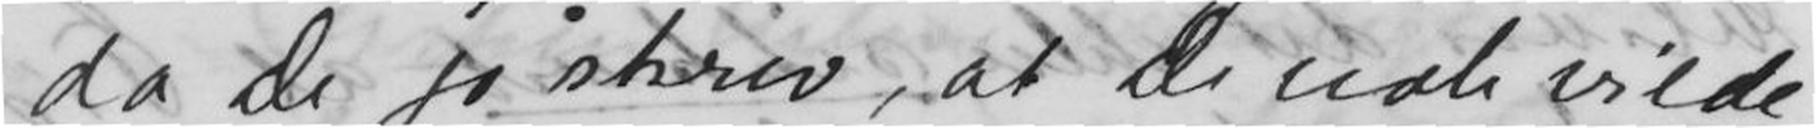da De jo skrev, at De nok vilde
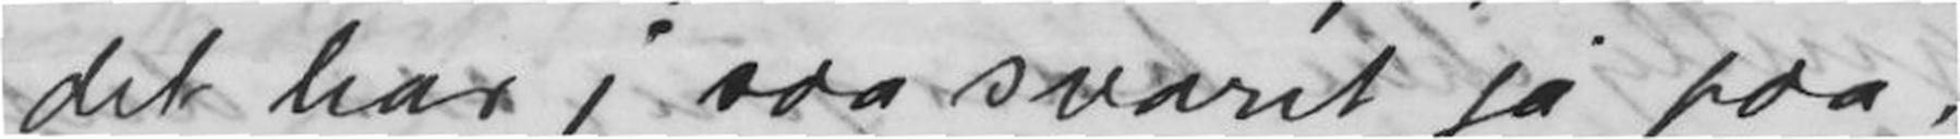det har j saa svaret ja paa,
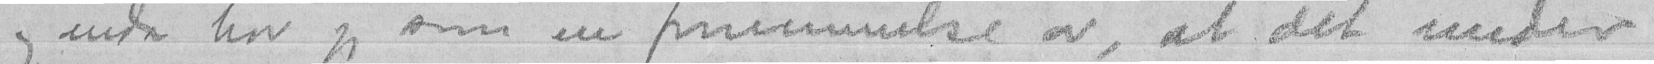og enda har jeg en fornemmelse av, at det under
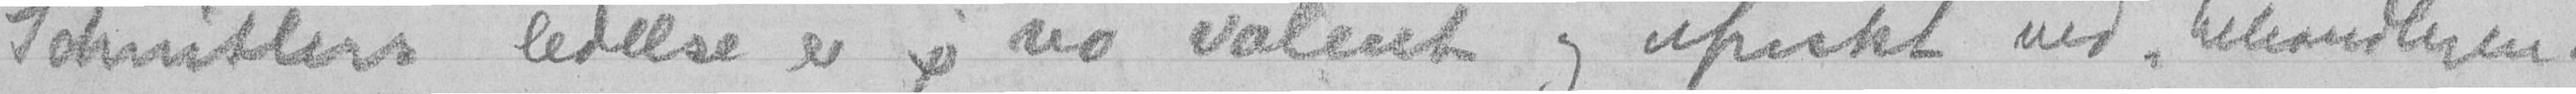Schnitlers ledelse er i no valent og ufriskt ved „behandlingen”.
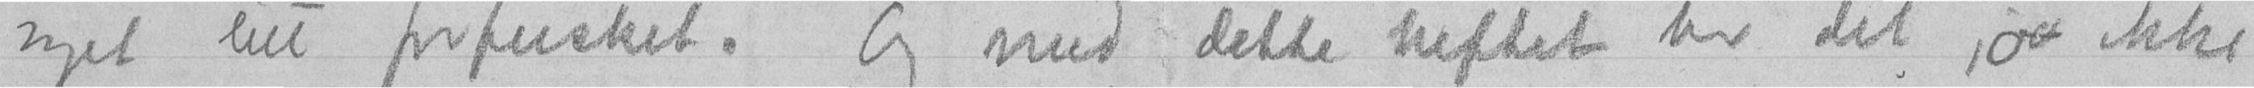noget litt forfusket. Og med dette heftet har det jo ikke
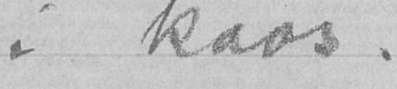i kaos.
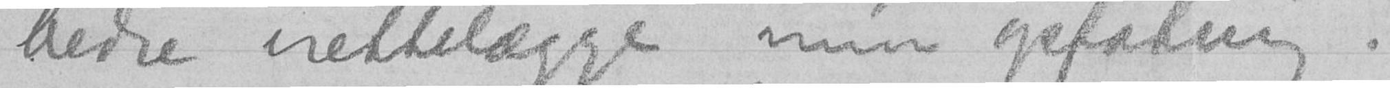bedre irettelægge min opfatning.
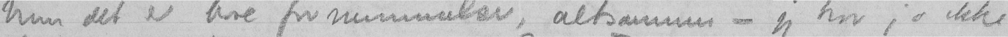Men det er bare fornemmelser, altsammen - jeg har jo ikke
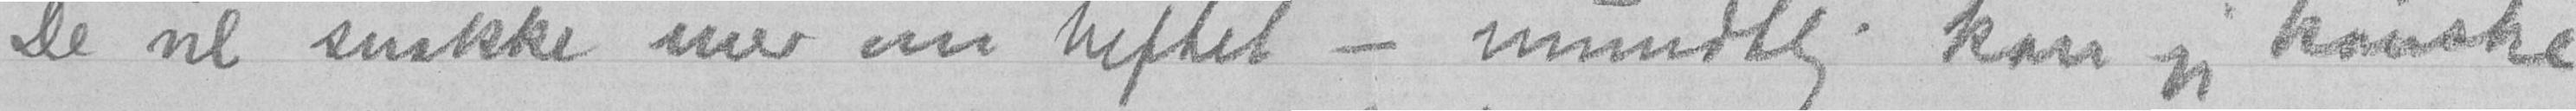De vil snakke mer om heftet - muntlig kan jeg kanske
